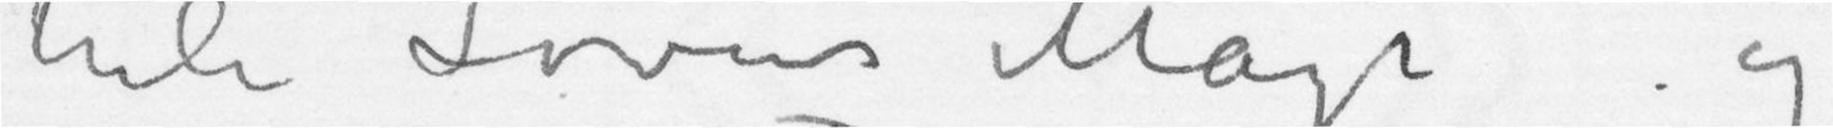hele Lovens Magt og
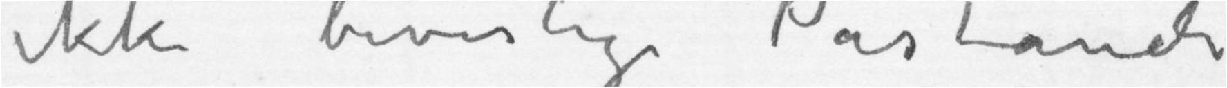ikke beviselige Påstande
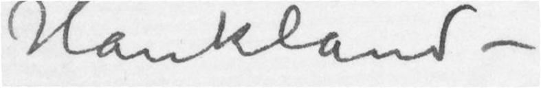Haukland –
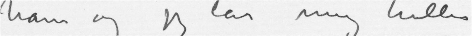ham og jeg lar mig heller
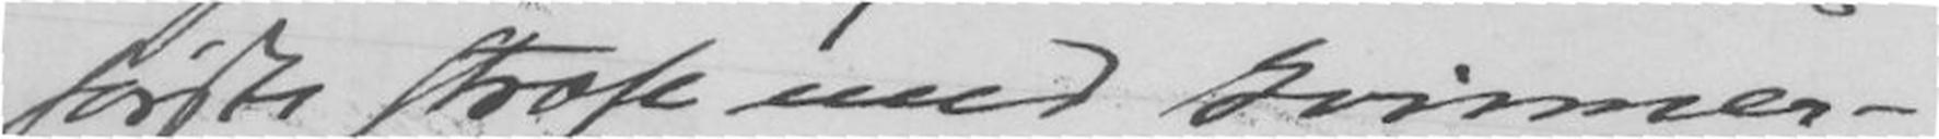første strofe med kvinner-
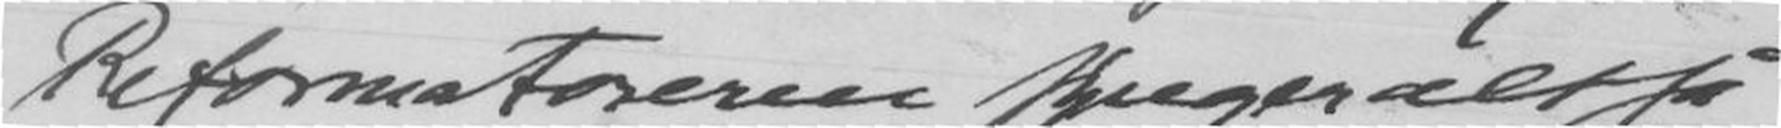Reformatorerne synger altså
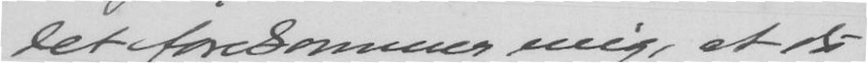Det forekommer mig, at du
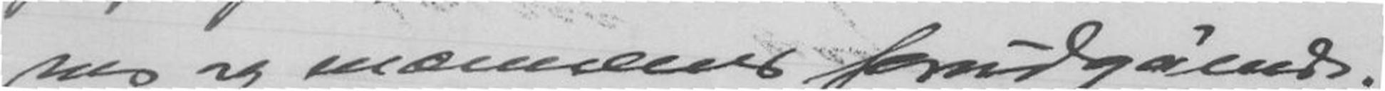nes og mænnenes forudgående.
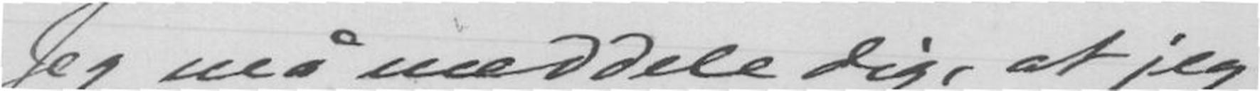Jeg må meddele dig, at jeg
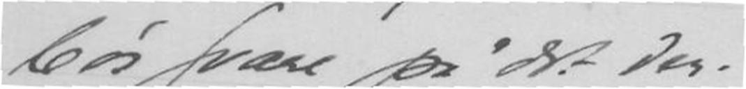bør svare på det der.
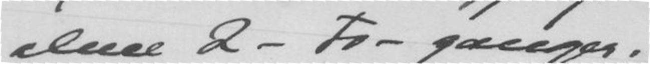alene 2 - to - ganger.
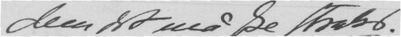Men det må ske straks.
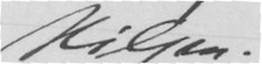Hilsen!
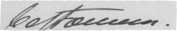bestæmme.
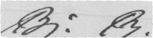Bj. Bj.
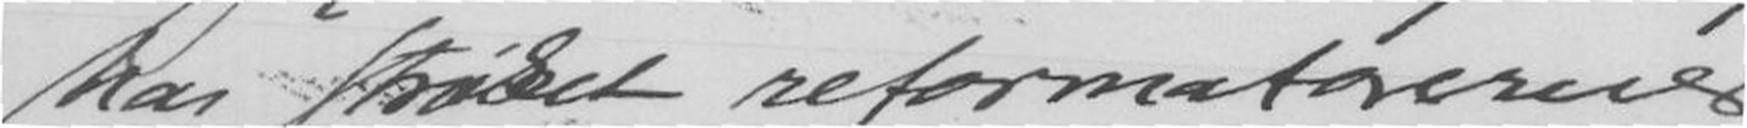har strøket reformatorernes
